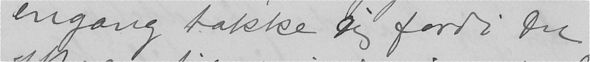engang takke Dig fordi Du
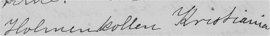Holmenkollen Kristiania
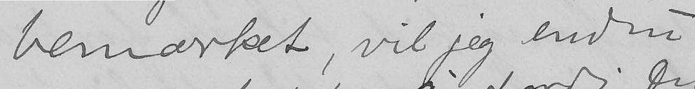bemærket, vil jeg endnu
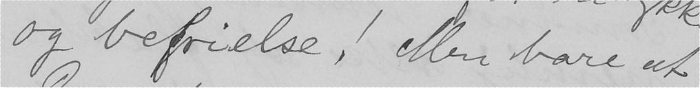og befrielse! Men bare at
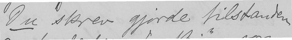Du skrev gjorde tilstanden
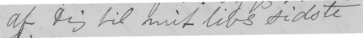at Dig til mit livs sidste
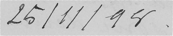25/11/98.
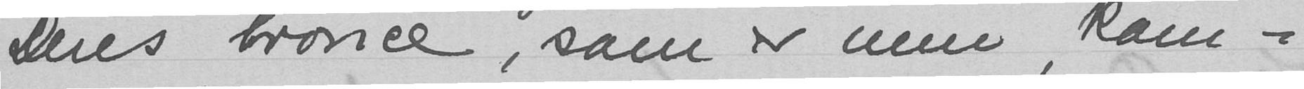Deres Bronce, som er min, kom-
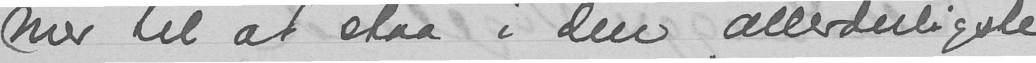mer til at staa i den allerdeiligste
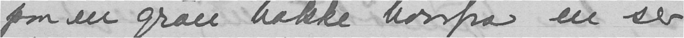paa en grøn bakke hvorfra en ser
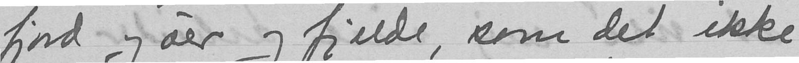fjord og øer og fjelde, som det ikke
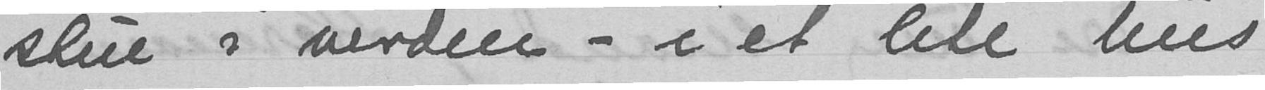stue i verden - i et lite hus
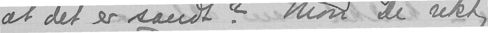at det er sandt? Mon De riktig
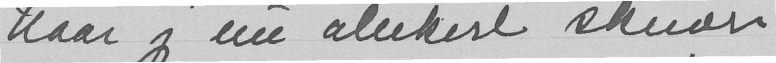Naar jeg nu allikevel skriver
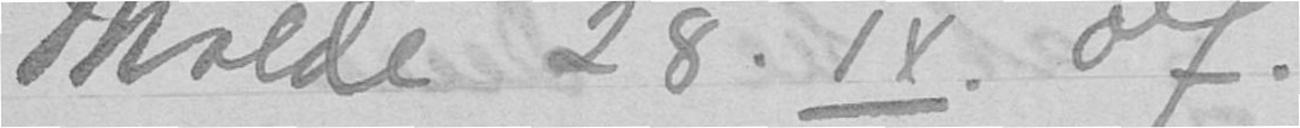Molde 28. IX. 07.
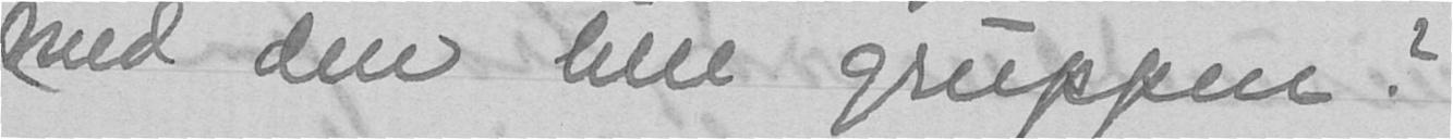med den lille gruppen?
